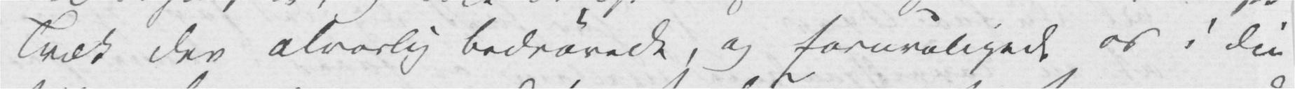Træk der alvorlig bedrøvede, og foruroligede os i Din
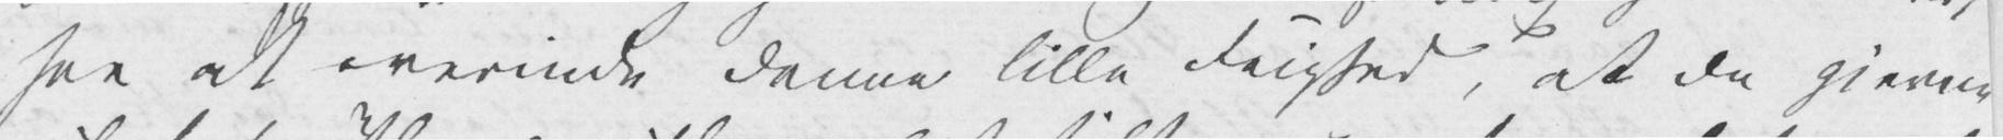see at overvinde denne lille Feighed, at Du gjerne
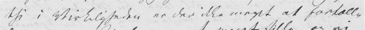thi i Virkeligheden er der ikke meget at fortælle
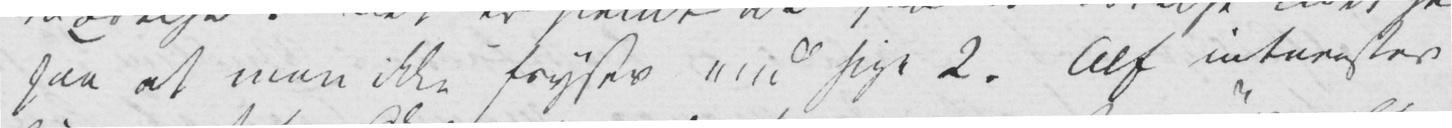saa at man ikke fryser end sige 2. Alf interesser
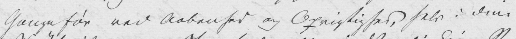Gange før ved Aabenhed og Oprigtighed, selv i Dine
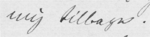mig tilbage.
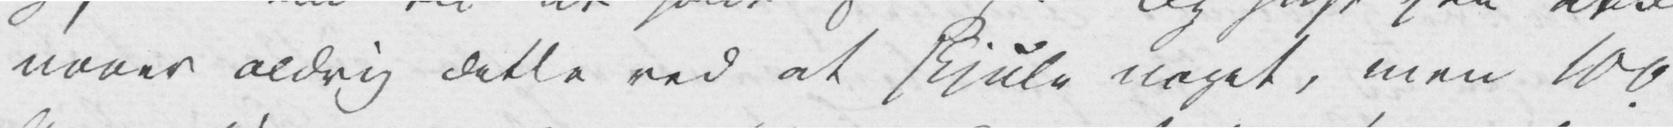naaer aldrig dette ved at skjule noget, men 100
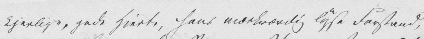kjerlige, gode Hjerte, hans mærkværdig lyse Forstand,
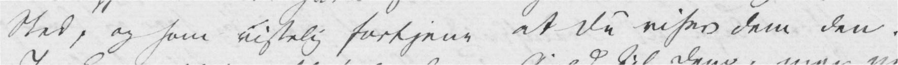Sted, og som visselig fortjene at Du viser dem den.
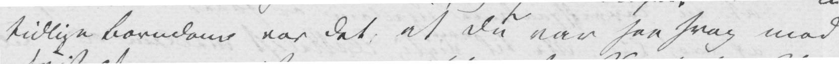tidlige Barndom var det, at Du var saa svag mod
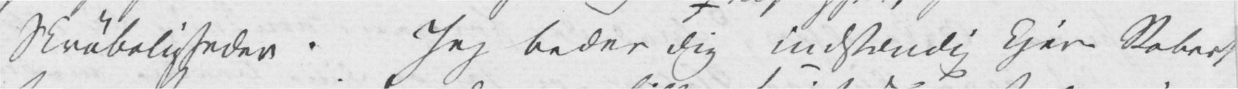Skrøbeligheder. Jeg beder Dig indstændig kjere Robert
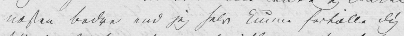næsten bedre end jeg selv kunne fortælle Dig,
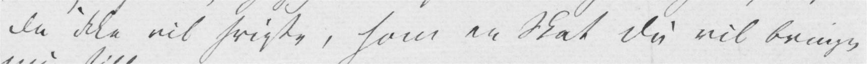Du ikke vil svigte, som en Skat Du vil bringe
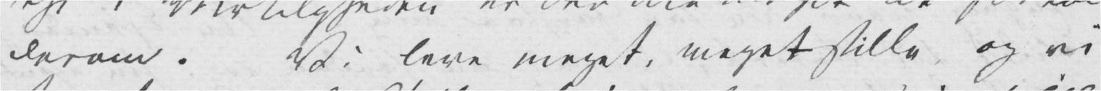derom. Vi leve meget, meget stille, og vi
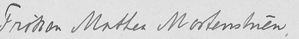Frøken Mathea Mortenstuen,
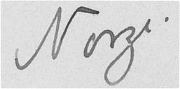Norge.
